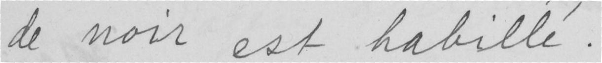de noir est habille.
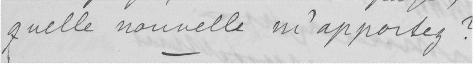quelle nouvelles m'apportez?
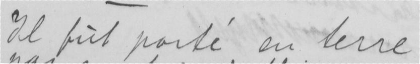Il ful porté en terre
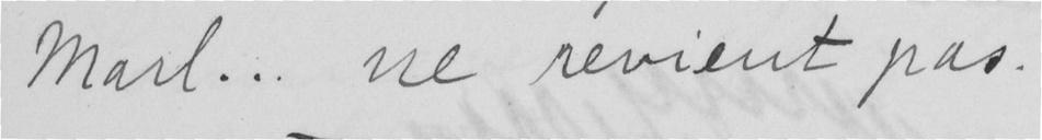Marl... ne revient pas.
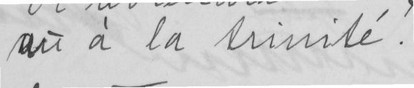nu á la trinite.
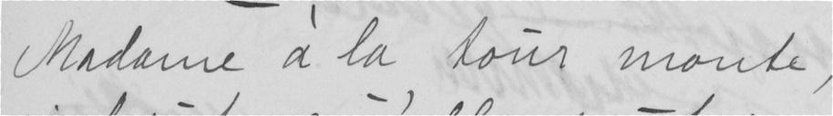Madame á la tour monte
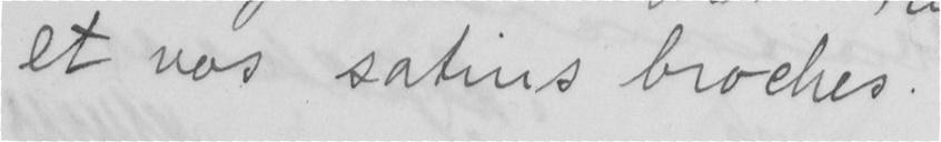et vos satins broches.
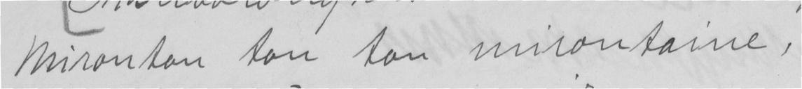Mironton ton ton mirontaine,
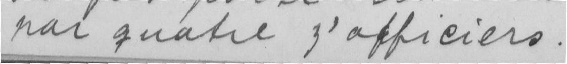har quatre z'officiers.
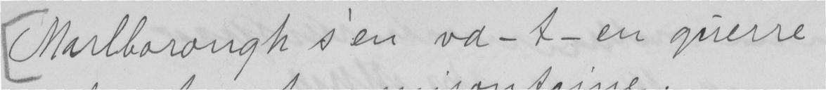Marlboroughs'en va-t-en guerre
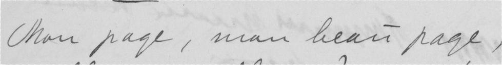Mon page, man bean page,
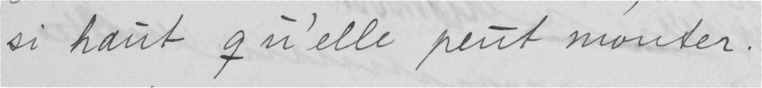si haut qu'elle peut monter.
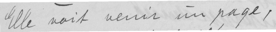Elle noit venir un page,
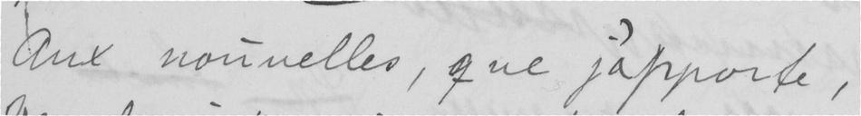Anx nouvelles, que j'apporte,
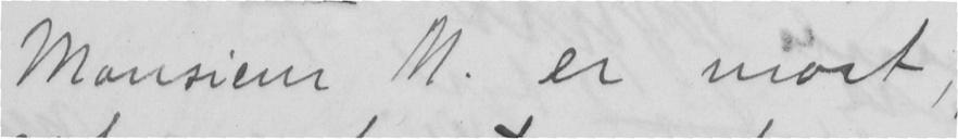Monsieur M. er mort,
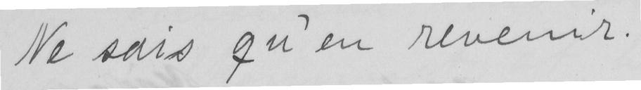Ne sais qu'en revenir.
